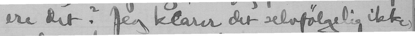ere det? Jeg klarer det selvfølgelig ikke,
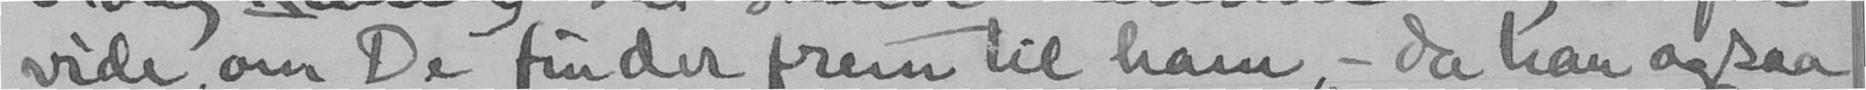vide, om De finder frem til ham - da han ogsaa
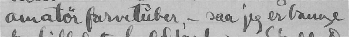amatør farvetuber, - saa jeg er bange
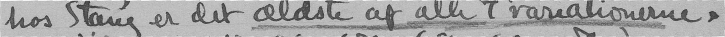hos Stang er det ældste af alle i variationerne.
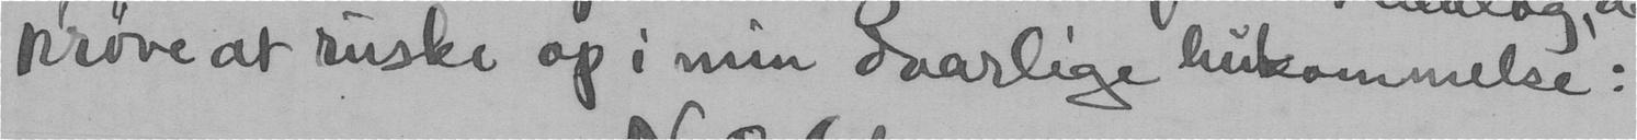prøve at ruske op i min daarlige hukommelse:
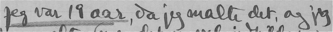Jeg var 19 aar, da jeg malte det, og jeg
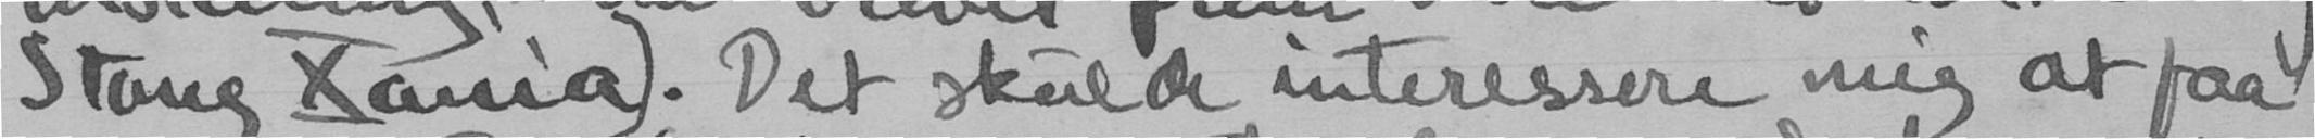Stang Xania). Det skulde interessere mig at faa
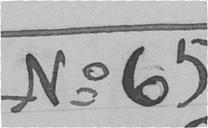N0 65
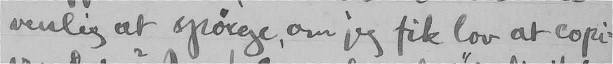venlig at spørige, om jeg fik lov at copi-
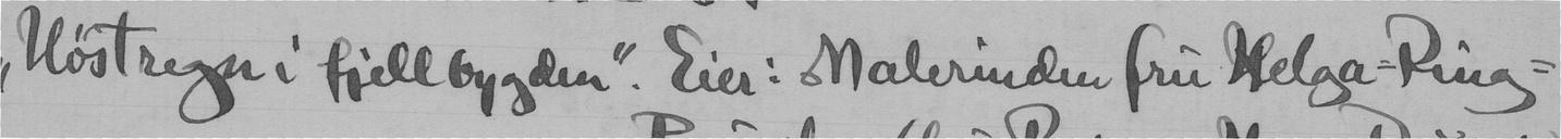"Høstregn i fjellbygden". Eier: Malerinden fru Helga Ring-
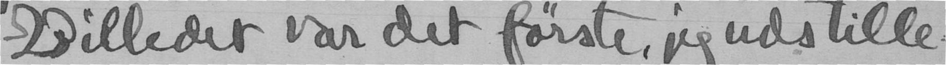Billedet var det første, jeg udstille-
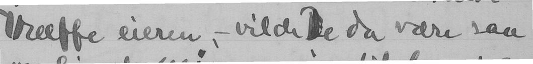treffe eieren, - vilde De da være saa
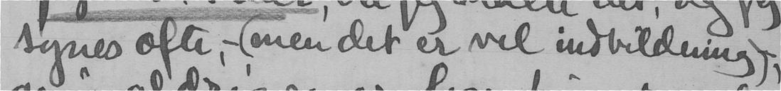synes ofte, - (men det er vel indbildning);
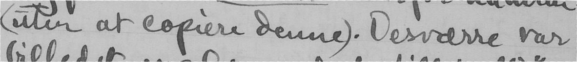(uten at copiere denne). Desværre var
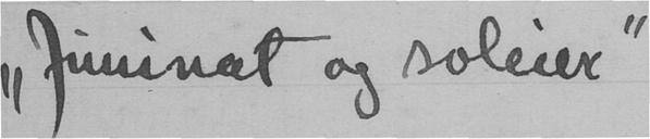"Juninat og soleier"
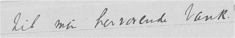til min herværende bank.
